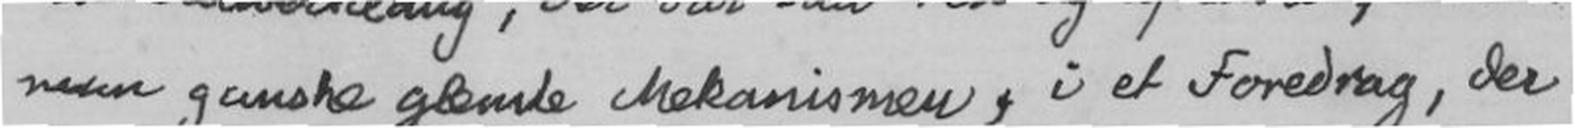man ganske glæmte Mekanismen, i et Foredrag, der
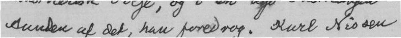saadan af det, han foredrog. Kurt nissen
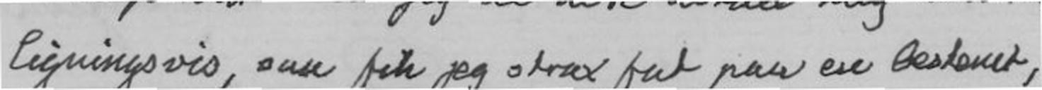ligningsvis, saa fik jeg strax fat paa en bestemt,
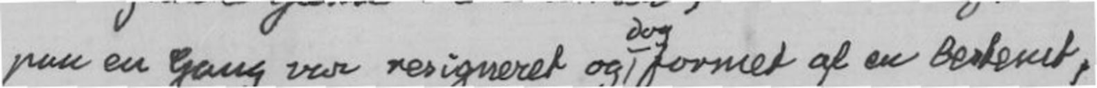paa en Gang var resigneret og dog formet af en bestemt,
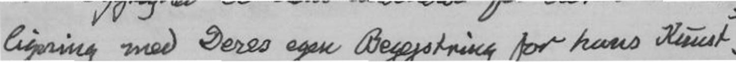ligning med Deres egen Begejstring for hans Kunst.
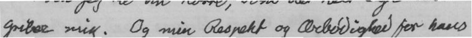griber mig. Og min Respekt og Ærbødighed for hans
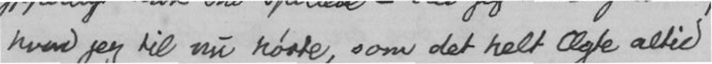hvad jeg til nu hørte, som det helt Ægte altid
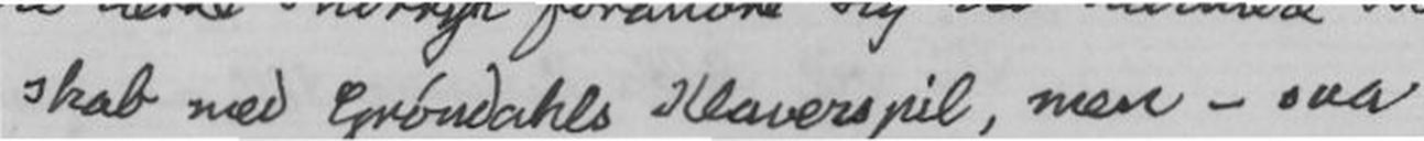skab med Grøndahls Klaverspil, men - saa
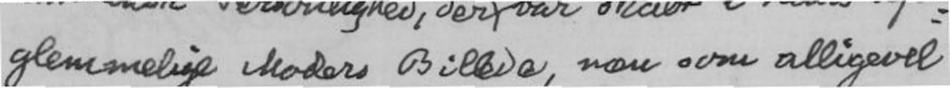glemmelige Moders Billede, noe som allikervel
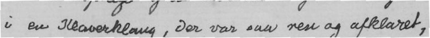i en klaverklang, der var saa ren og afklaret,
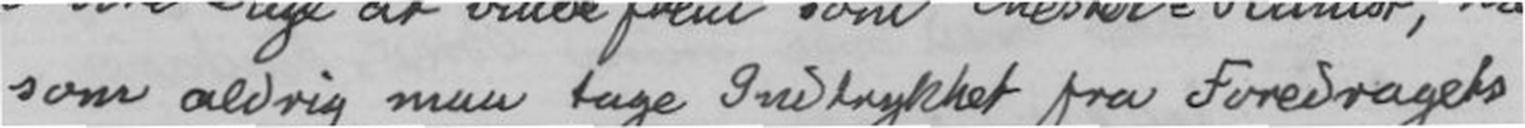som aldrig maa tage Indtrykket fra Foredragets
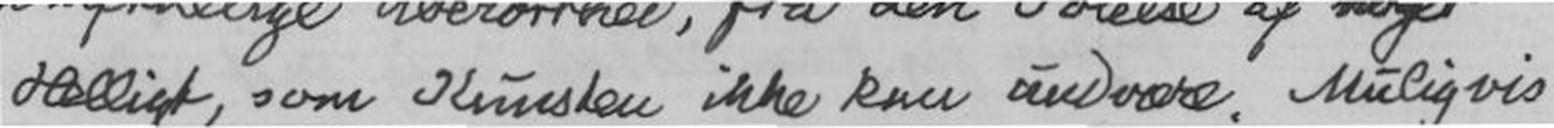Helligt, som Kunsten ikke kan undvære. Muligvis
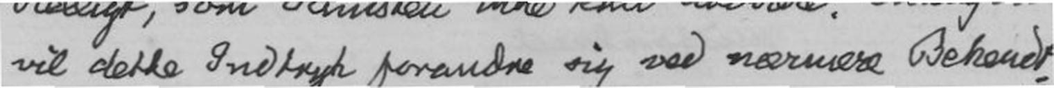vil dette Indtryk forandre sig ved nærmeræ Bekendt-
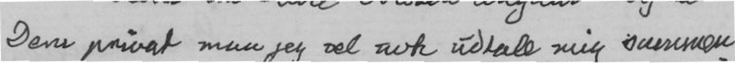Dem privat maa jeg vel nok udtale mig sammen-
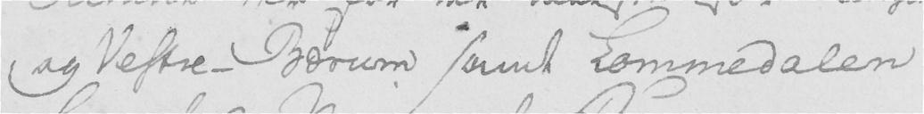og Vestre-Bærum samt Lommedalen
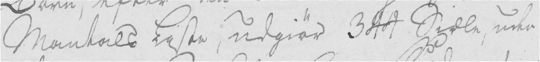Mantals Liste, udgiør 344 Siæle, uden
